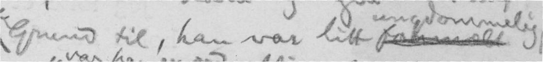Grund til, han var litt faamælt
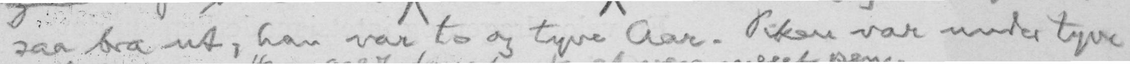saa bra ut, han var to og tyve Aar. Piken var under tyve
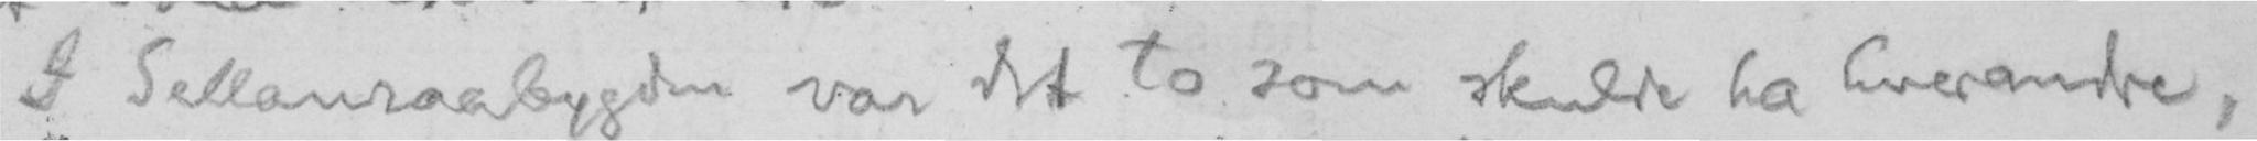I Sellanraabygden var det to som skulde ha hverandre,
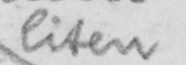liten
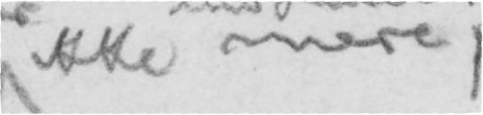ikke mere
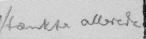tænkte allerede
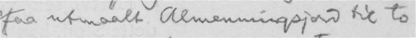faa utmaalt Almenningsjord til to
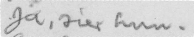Ja, sier hun.
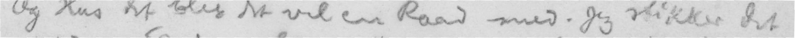Og Hus det blir det vel en Raad med. Jeg stikker det
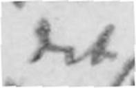det
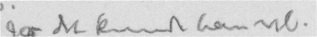Jo det kunde han vel.
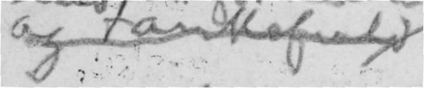og tankefuld
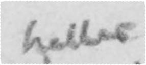heller
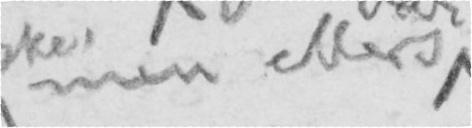men ellers
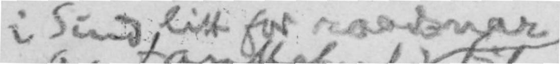i Sind, litt for raadsnar
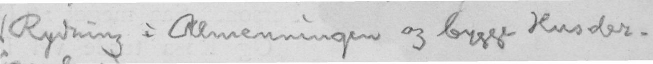Rydning i Almenningen og bygge Hus der.
